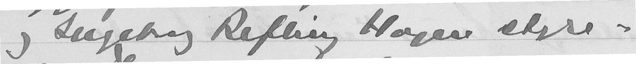og Ingeborg Refling Hagen styre-
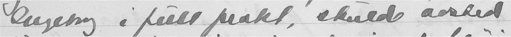Ingeborg i full prakt, skulde avsted
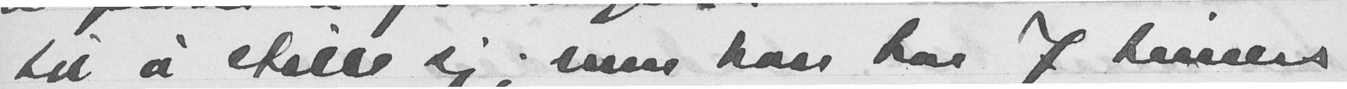til å stille sig; men han har 7 timers
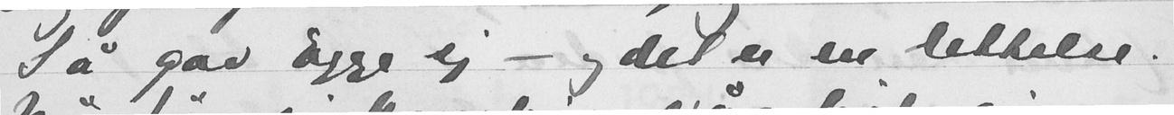Så gav Egge sig - og det er en lettelse.
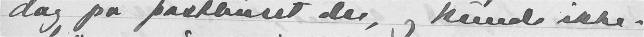dag på posthuset der, og kunde ikke.
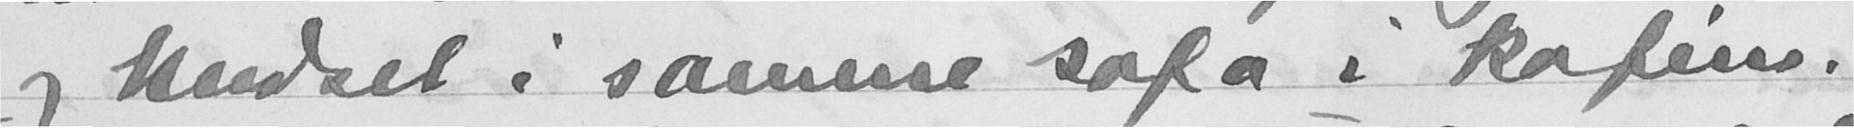og Undset i samme sofa i kaféen.
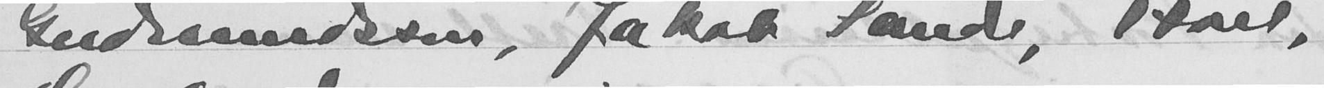Gudmundsson, Jakob Sande, Hoel,
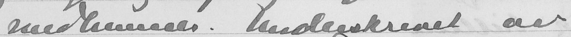medlemmer. Underskrevet av
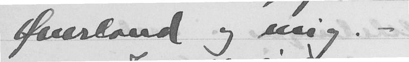Øverland og mig. -
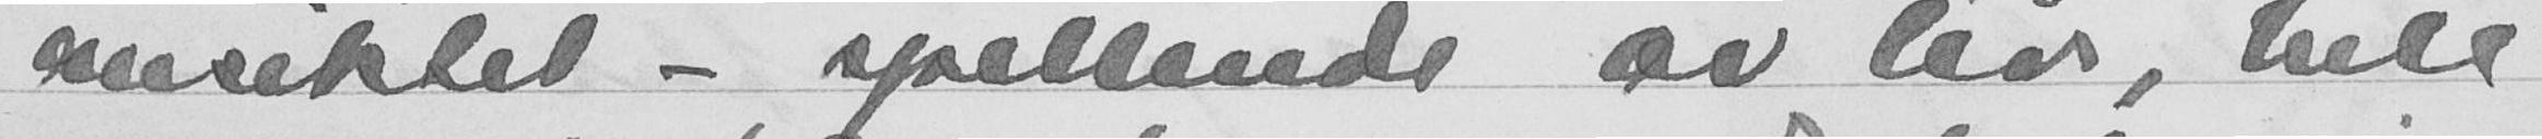musikken - spillende av liv, hele
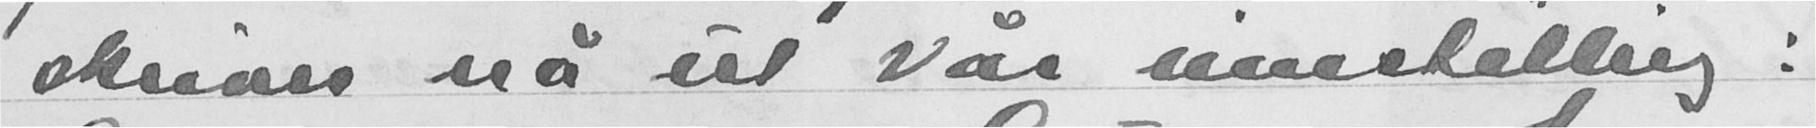skriver nå til vår innstilling!
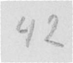42
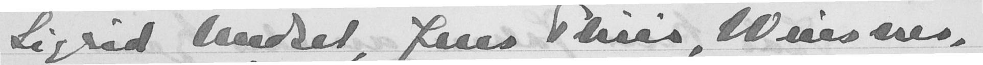Sigrid Undset, Jens Thiis, Winsnes,
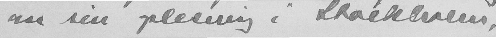om sin oplesning i Stockholm,
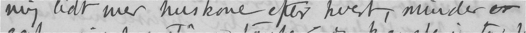mig lidt mer huskone efter hvert, minder er
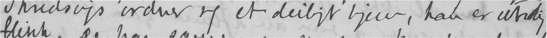Skredsvigs ordner sig et deiligt hjem, han er utrolig
